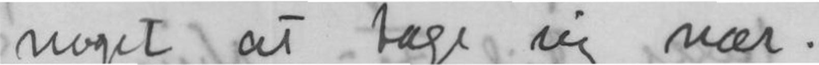noget at tage sig nær.
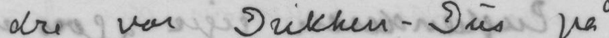dre vor Drikken Dus på
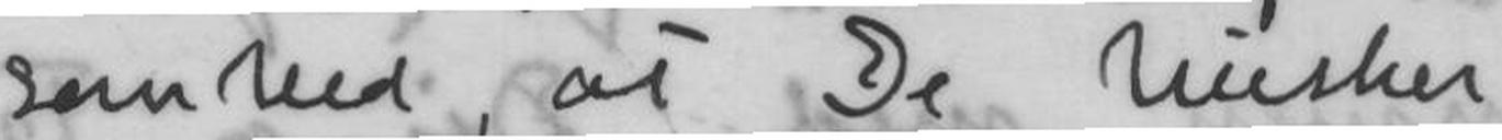somhed, at De husker
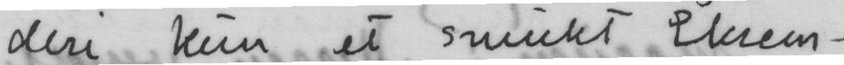deri kun et smukt Eksem-
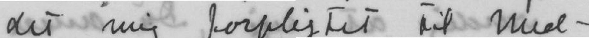det mig forpligtet til Med-
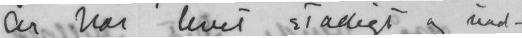År har levet stadigt og uad-
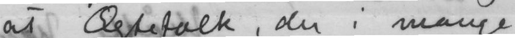at Ægtefolk, der i mange
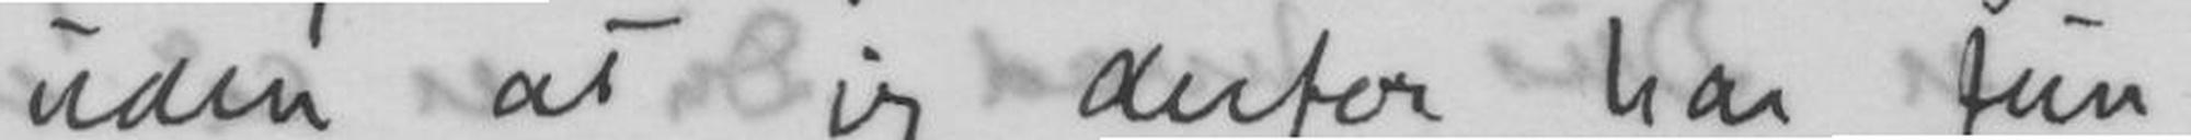uden at jeg derfor har fun-
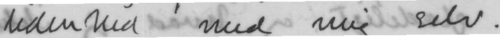lidenhed med mig selv.
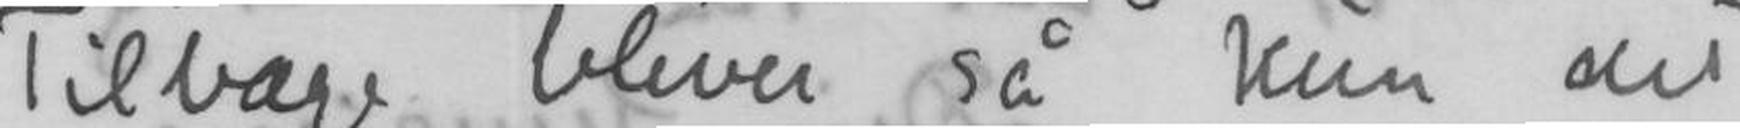Tilbage bliver så kun det
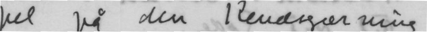pel på den Kendsgærning
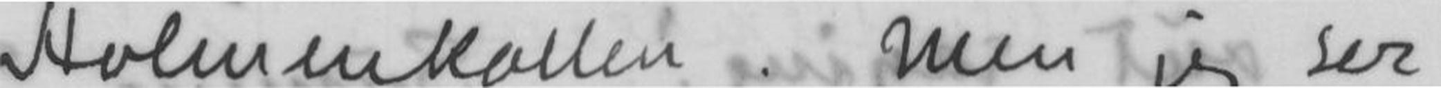Holmenkollen. Men jeg ser
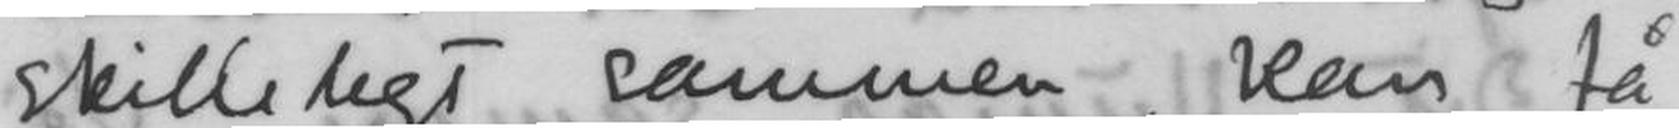skilligt sammen kan få
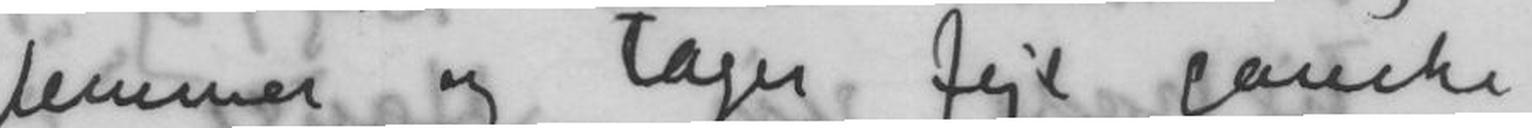glemmer og Tage fejl ganske
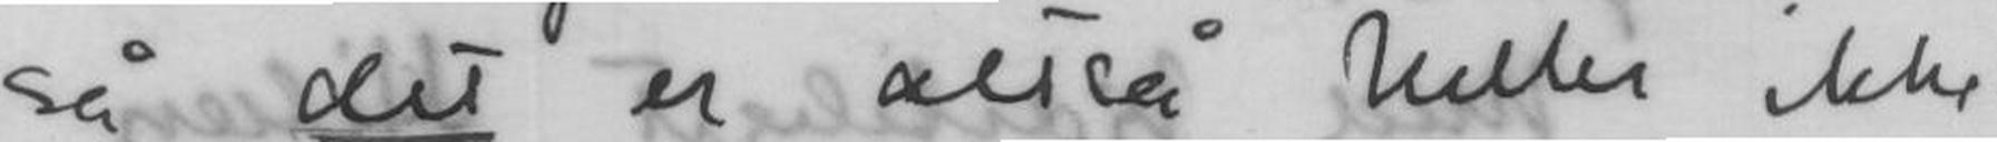så det er altså heller ikke
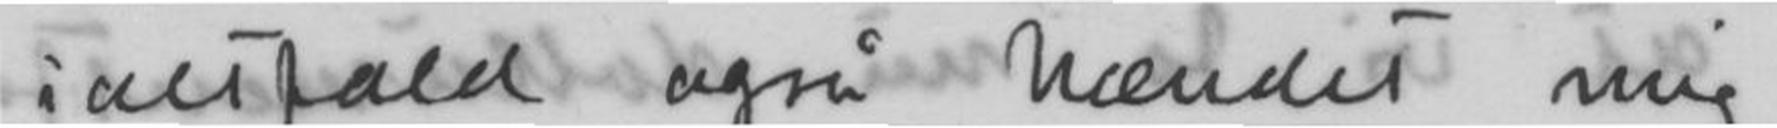ialtfald også hændet mig
